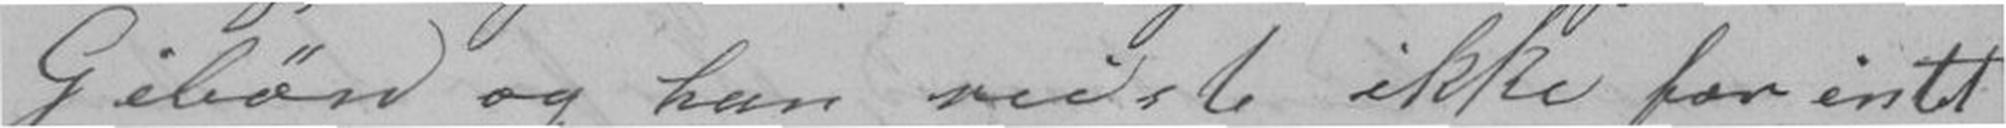Gibøn og han reiste ikke for intet
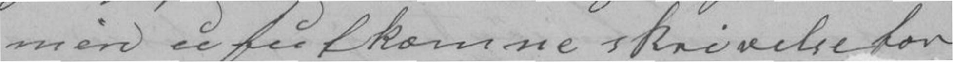min ufulkomne skrivelse for
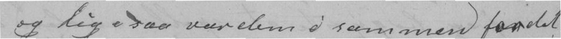og ligesaa var dem i sammen for det
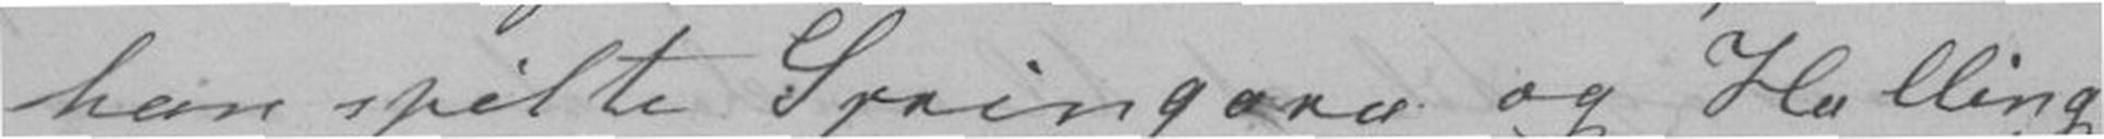han spilte Springara og Halling
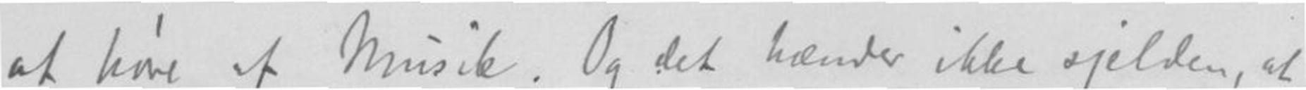at høre af Musik. Og det hænder ikke sjelden, at
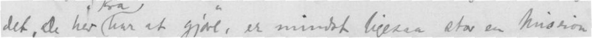har at gjøre, er minst ligesaa stor en Mission
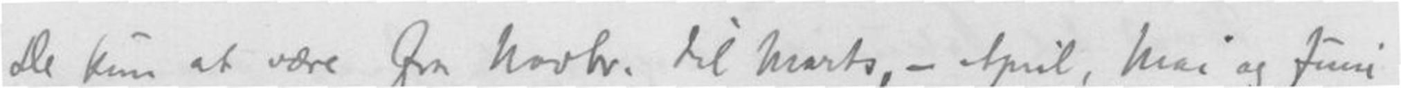de kun at være fra Novbr. til Marts,-April, Mai og Juni
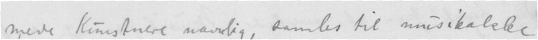mede Kunstnere nærlig, samles til musikalske
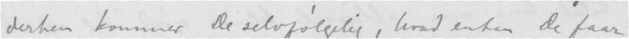derhen kommer De selvfølgelig, hvad enten De faar
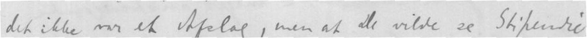det ikke var et Afslag, men at De vilde se Stipendie-
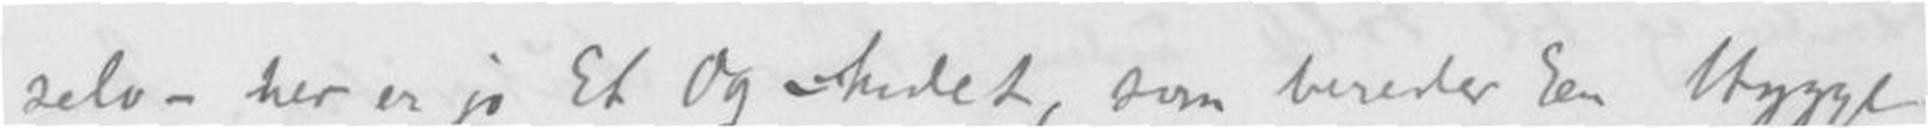selv- her er jo Et Og Andet, som bereder En Hygge
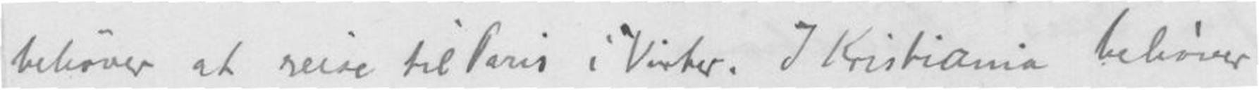behøver at reise til Paris i Vinter. I Kristiania behøver
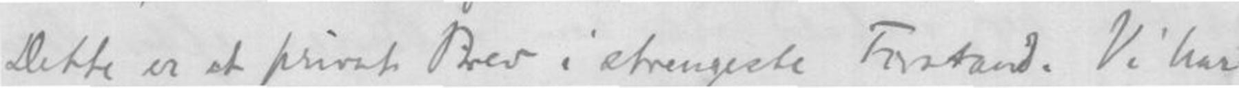Dette er et privat Brev i strengeste Forstand. Vi har
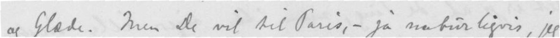og Glæde. Med De vil til Paris,- ja naturligvis, jeg
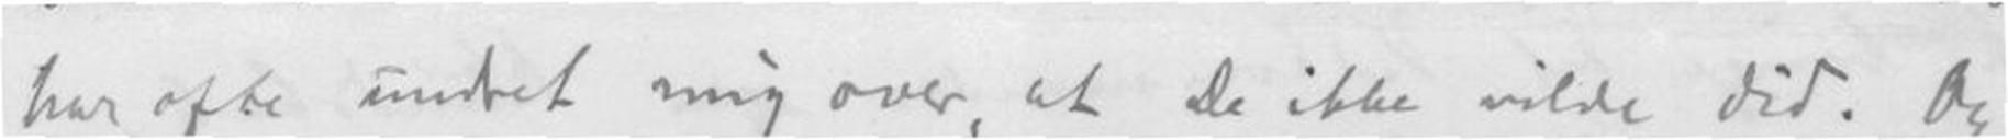har ofte undret mig over, at de ikke vilde dit. Og
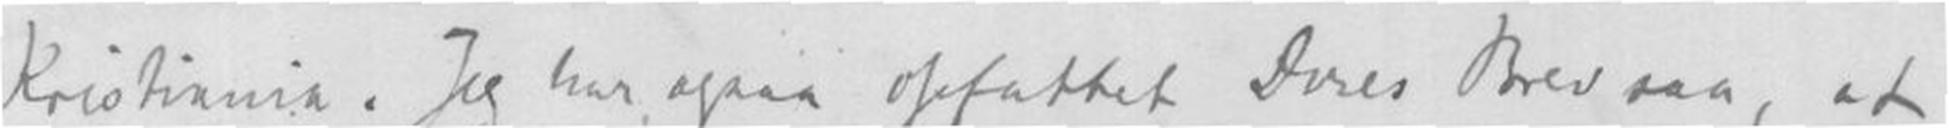Kristiania. Jeg har ogsaa opfattet Deres Brev saa, at
